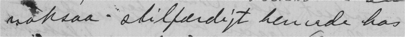noksaa stilfærdigt hernede hos
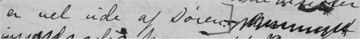er vel ude af Døren.
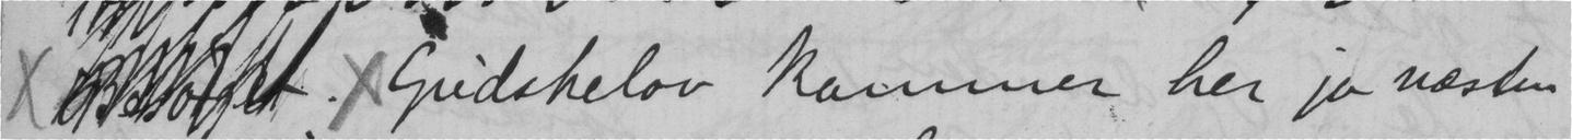. x Gudskelov kommer her jo næsten
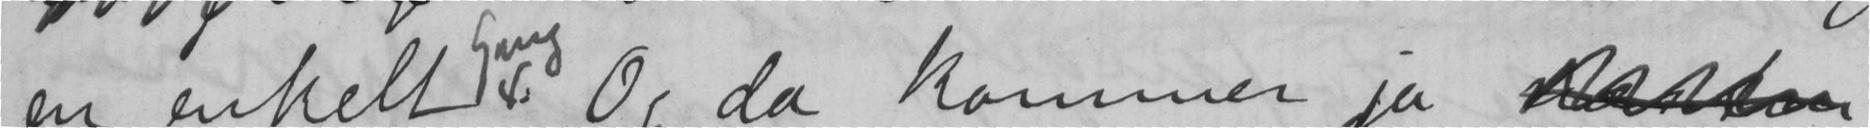en enkelt gang og da kommer jo
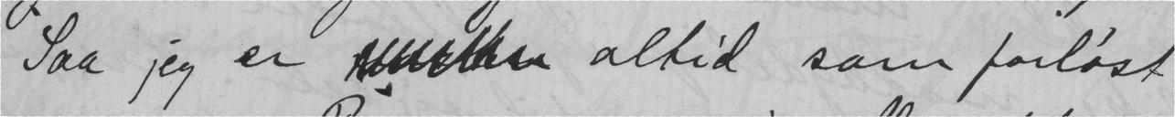Saa jeg er  altid som forløst
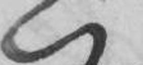G
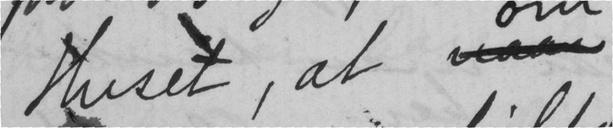Huset, at
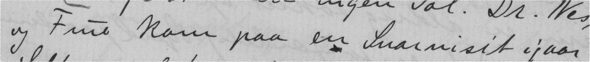og Frue kom paa en Snarvisit igaar
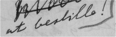at bestille!
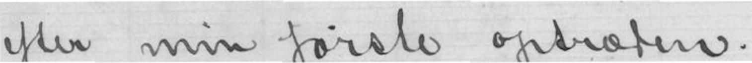efter min første optræden.
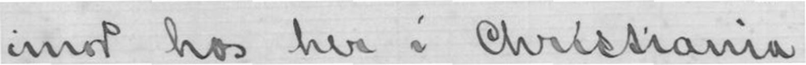imod hos her i Christiania,
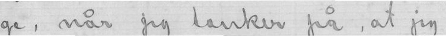ge, når jeg tænker på, at jeg
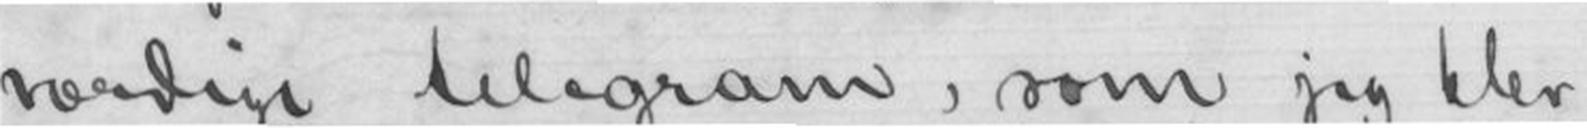værdige telegram, som jeg blev
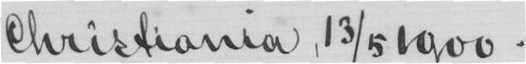Christiania, 13/5 1900.
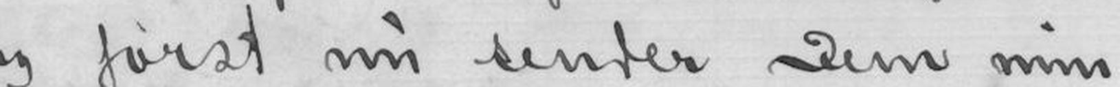jeg først nu sender Dem min
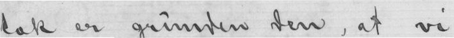tak er grunden den, at vi
